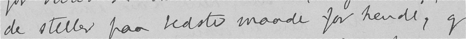de steller paa bedste maade for hende, og
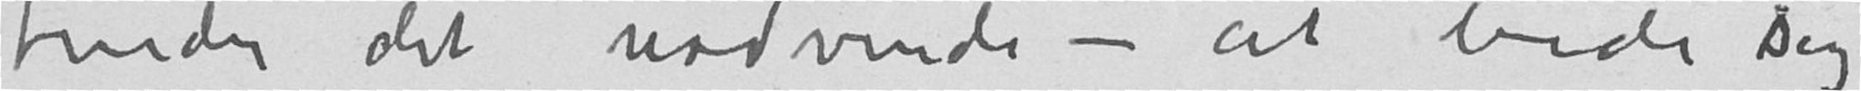finder det nødvendi – at bede Dig
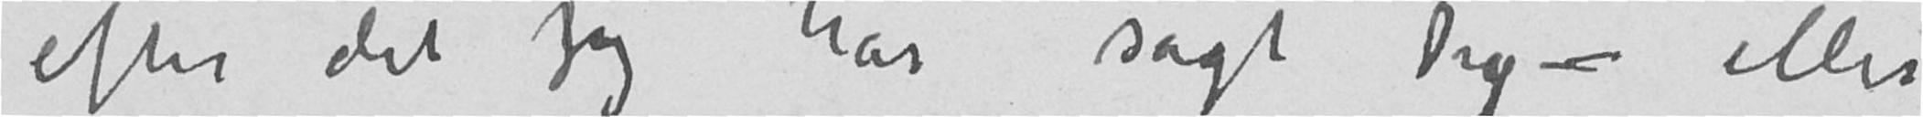efter det jeg har sagt Dig – eller
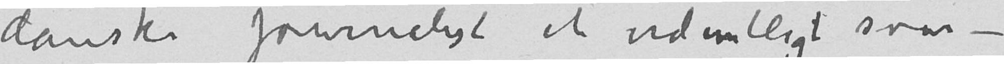danske journalist et ordentligt svar –
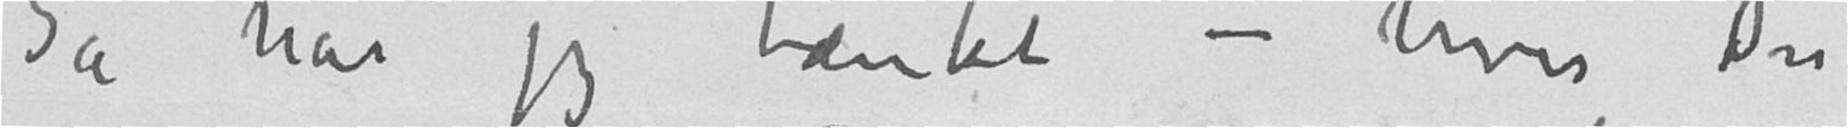Så har jeg tænkt – hvis Du
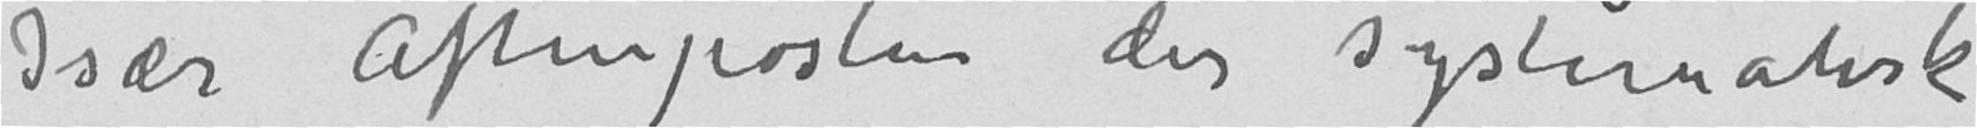Især Aftenposten der systematisk
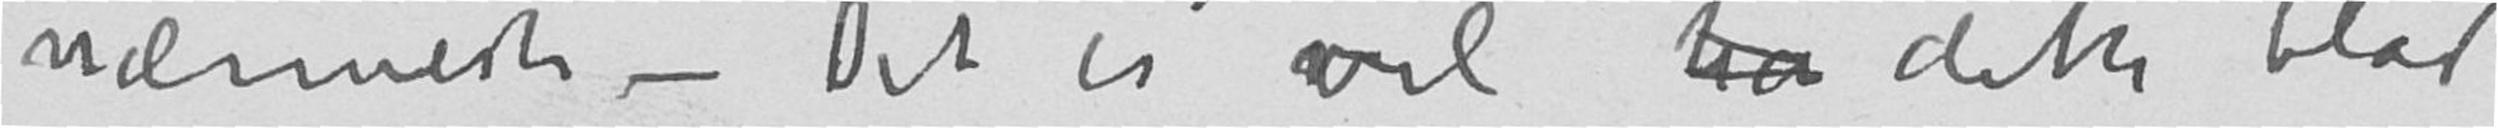nærmeste – Det er vel ha dette blad
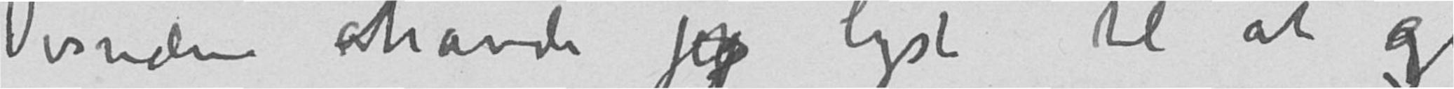Desuden ahavde jeg lyst til at gi
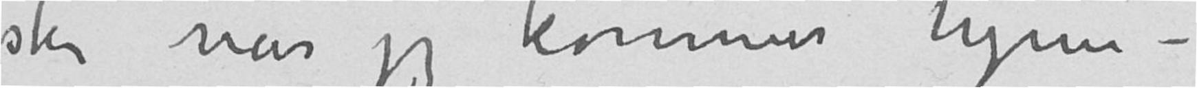ske når jeg kommer hjem –
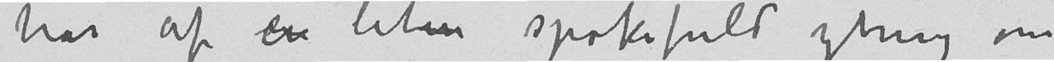har af en liden spøkefuld ytring om
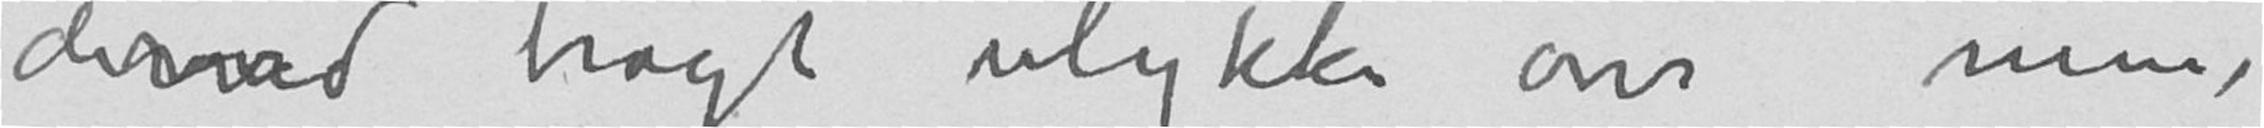derved bragt ulykke over mine
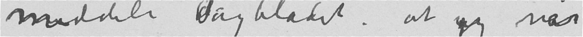meddele Dagbladet at jeg når
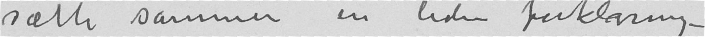sætte sammen en liden forklaring –
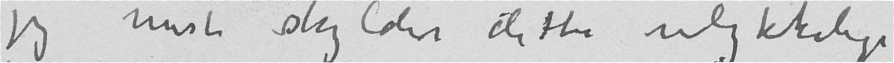jeg mest skylder dette ulykkelige
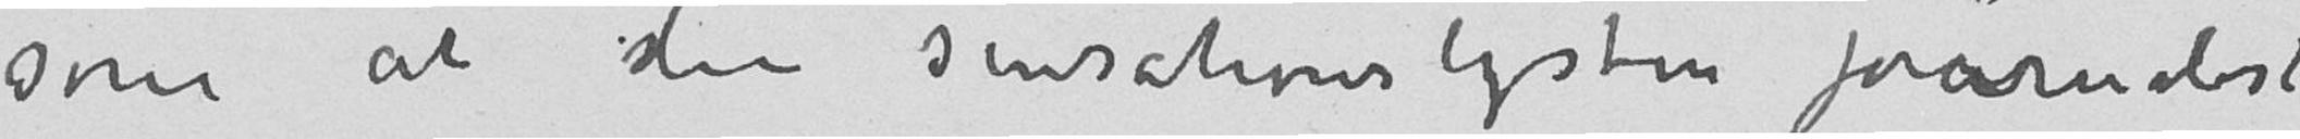som at den sensationslystne journalist
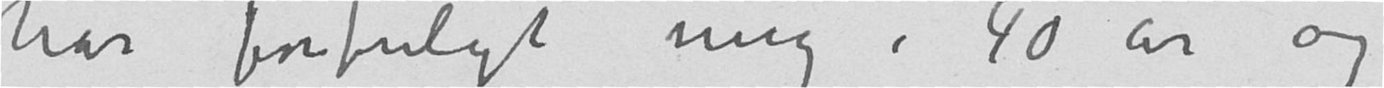har forfulgt mig i 40 år og
